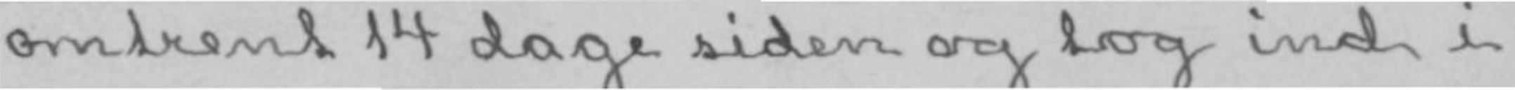omtrent 14 dage siden og tog ind i
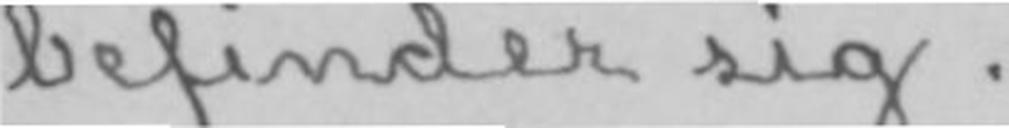befinder sig.
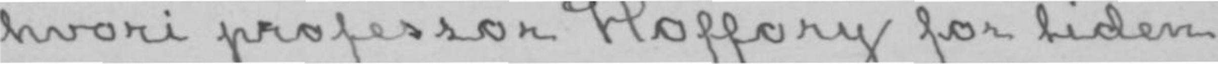hvori professor Hoffory for tiden
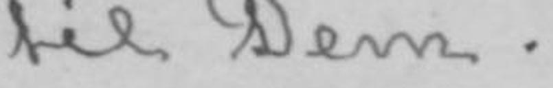til Dem.
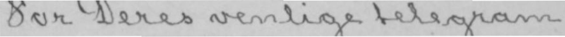For Deres venlige telegram
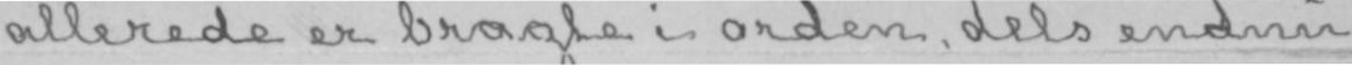allerede er bragte i orden, dels endnu
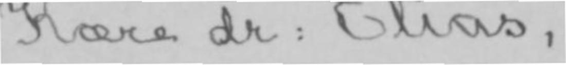Kære dr: Elias,
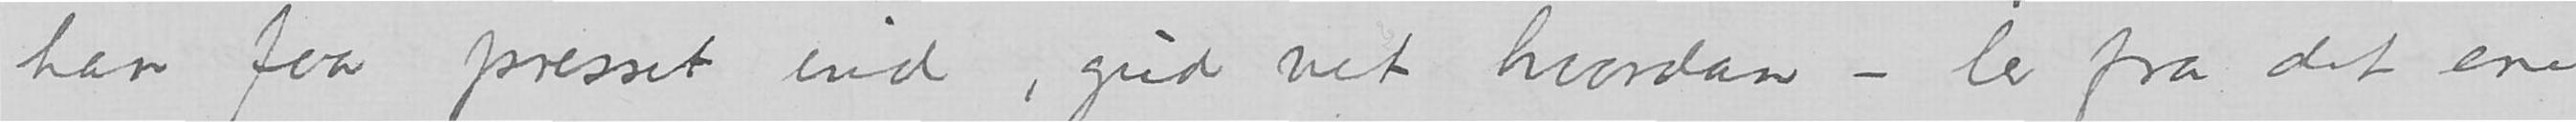han faar presset ind, gud vet hvordan – ler fra det ene
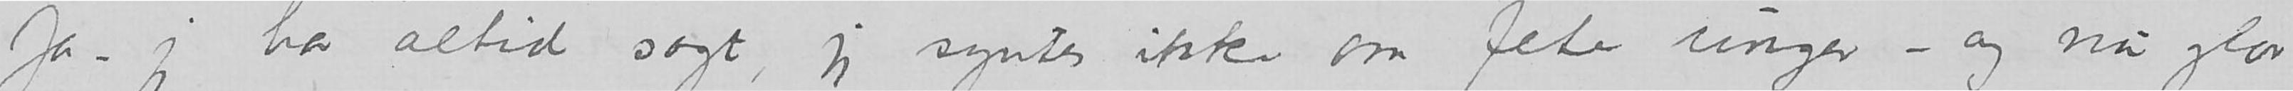Ja – jeg har altid sagt, jeg syntes ikke om fete unger – og nu glor
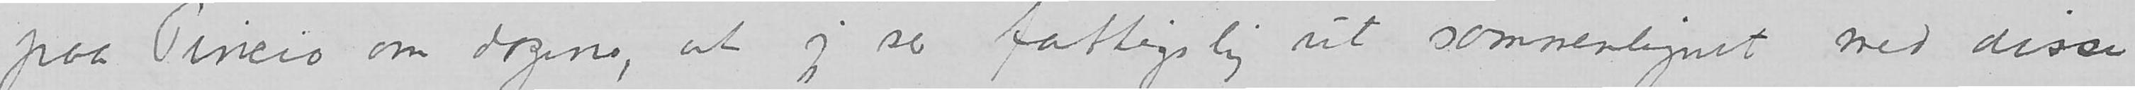paa Pincio om dagene, at jeg ser fattigslig ut sammenlignet med disse
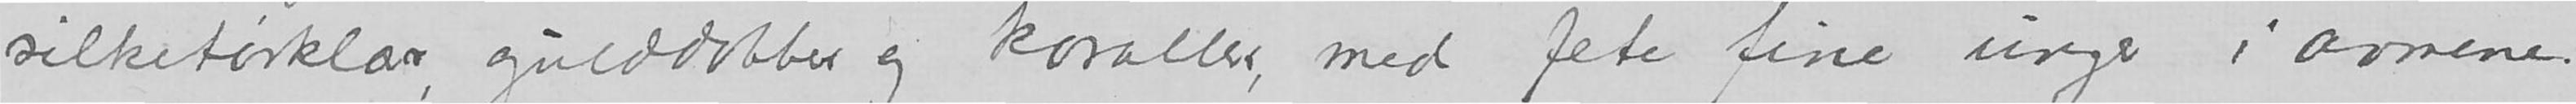silketørklær, gulddobber og koraller, med fete fine unger i armene.
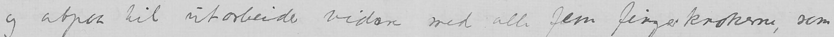og atpaa til utarbeider videre med alle fem fingerknrokerne, som
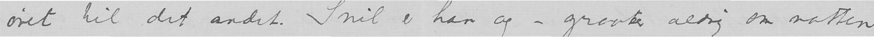øret til det andet. Snil er han og – graater aldrig om natten,
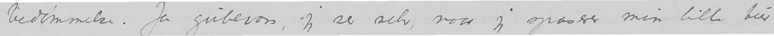bedømmelse. Ja gubevars, jeg ser selv, naar jeg spaserer min lille tur
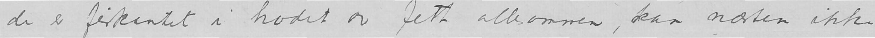de er firkantet i hodet av fett alletsammen, kan næsten ikke
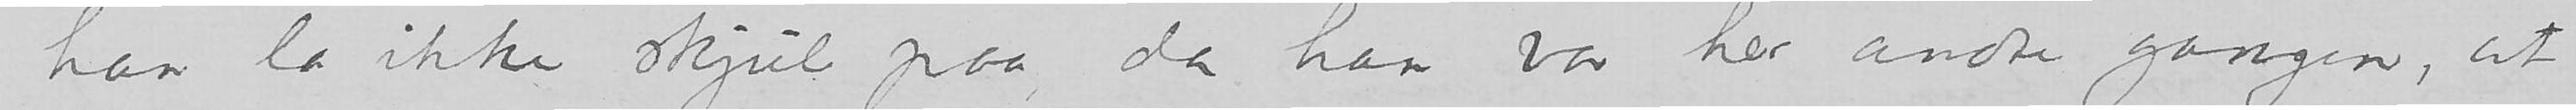–han la ikke skjul paa, da han var her andre gangen, at
